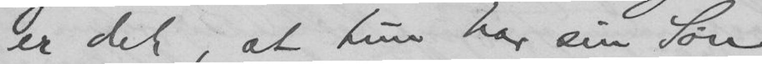er det, at hun har sin Søn
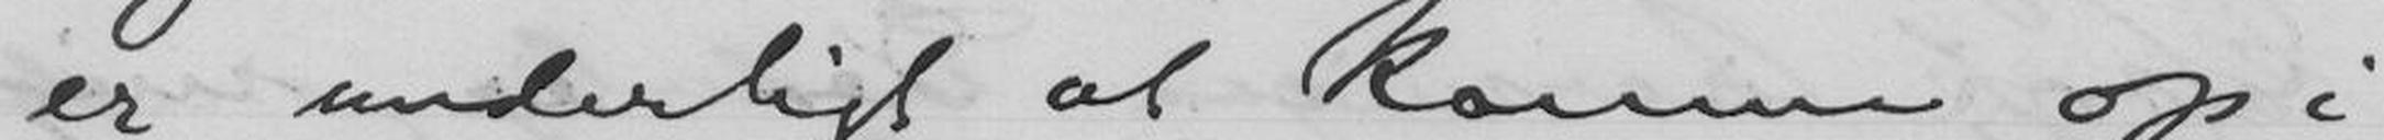er underligt at komme op i
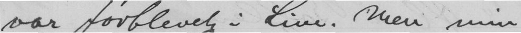var forblevet i Live. Men min
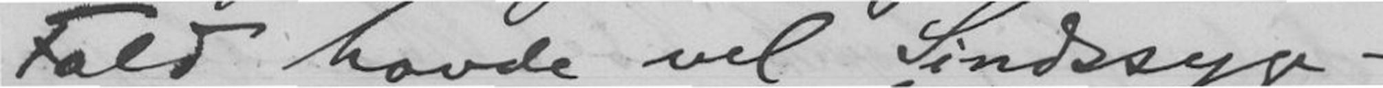Fald havde vel Sindsyge-
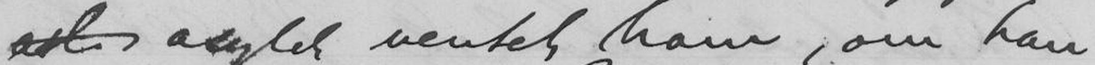asylet ventet ham, om han
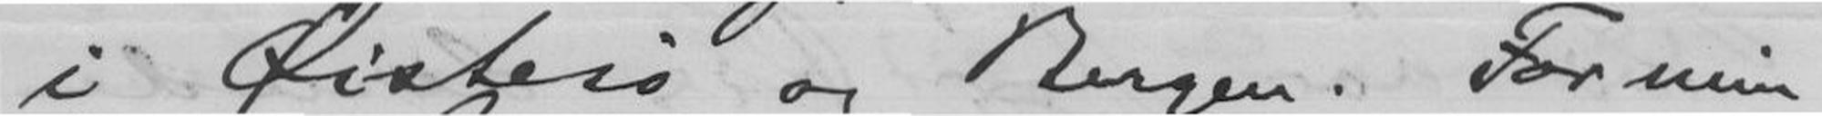i Øistesø og Bergen. For min
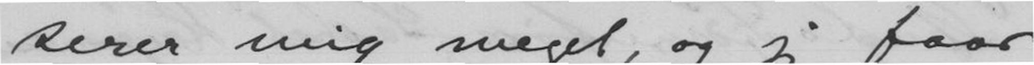serer mig meget, og jeg faar
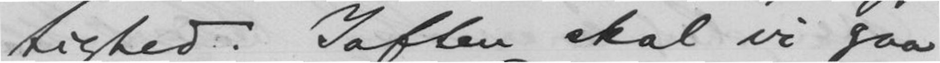tighed. Iaften skal vi gaa
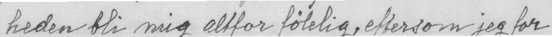heden bli mig altfor følelig, eftersom jeg for
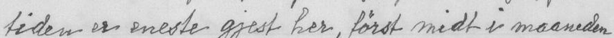tiden er eneste gjest her, først midt i maaneden
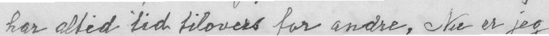har altid tid tilovers for andre. Nu er jeg
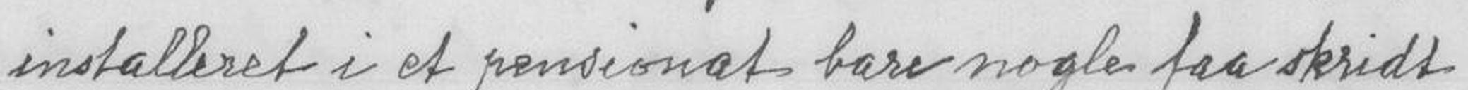installert i et pensionat bare nogle faa skrift
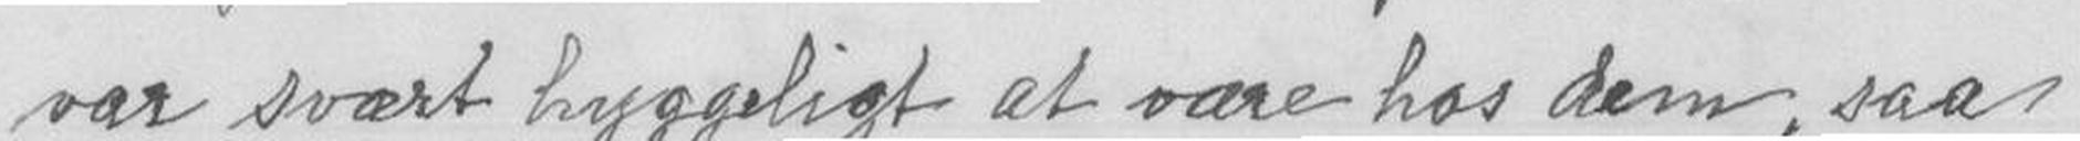var svært hyggeligt at være hos dem, saa
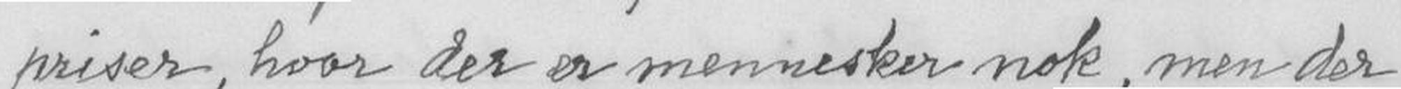priser, hvor der er mennesker nok, men der
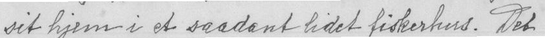sit hjem i et saadant lidet fiskerhus. Det
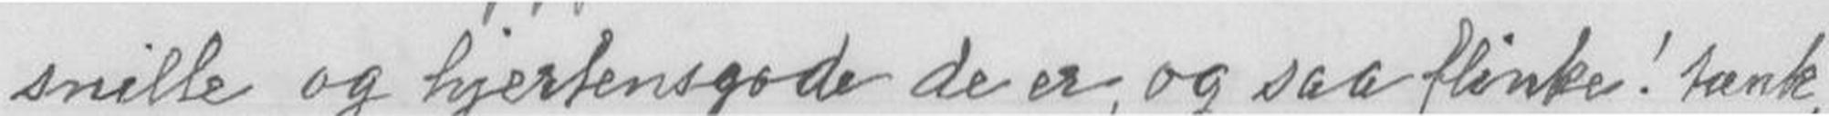snille og hjertensgode de er, og saa flinke! tænkte,
